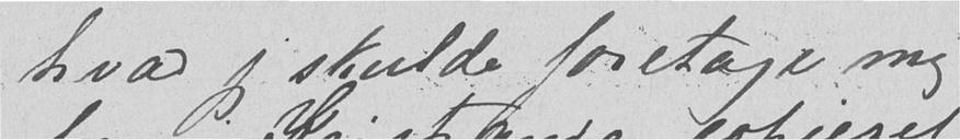hvad jeg skulde foretage mig
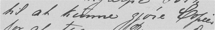til at kunne gjøre Copier
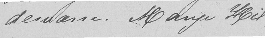desværre. Mange Hil-
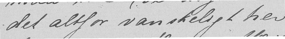det altfor vanskeligt her
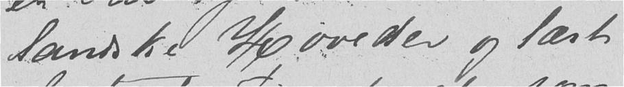landske Hoveder og lært
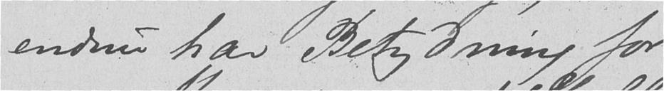endnu har Betydning for
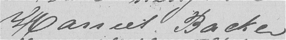Harriet Backer
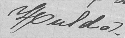Hulda.
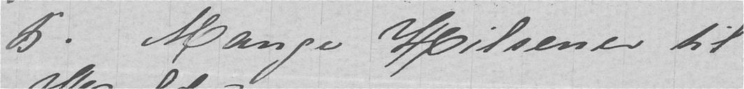jeg. Mange Hilsener til
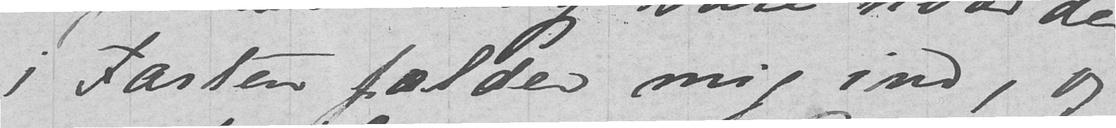i Farten falder mig ind, og
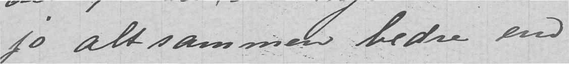jo alt sammen bedre end
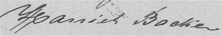Harriet Backer
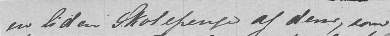en liden Skolepenge af dem, som
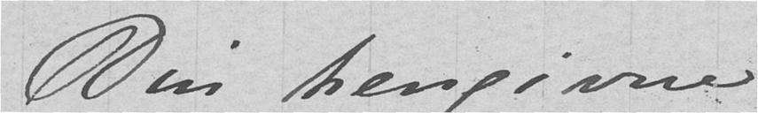Din hengivne
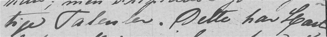tige Talenter. Dette har Hast
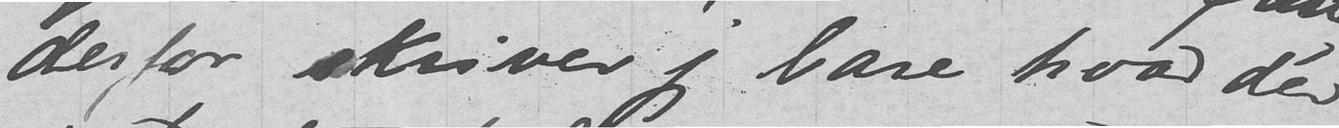derfor skriver jeg bare hvad der
